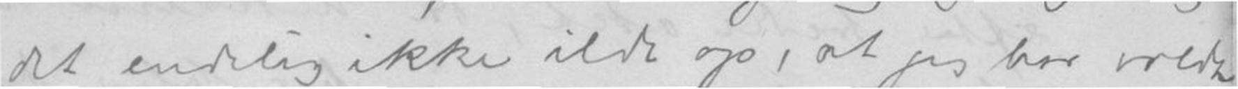det endelig ikke ilde op, at jeg har voldet
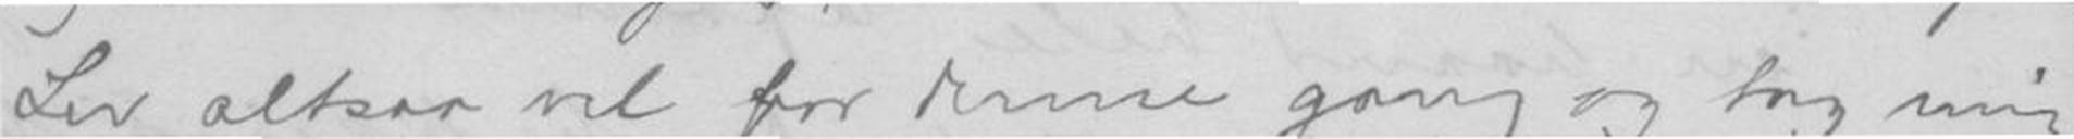Lev altsaa vel for denne gang og tag mig
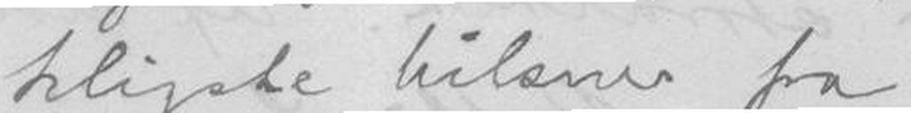teligste hilsner fra
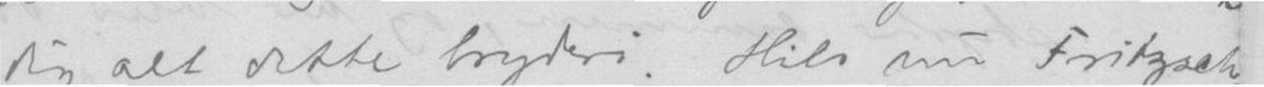dig alt dette bryderi. Hils nu Fritzsch
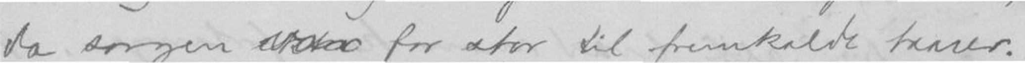da sorgen var for stor til fremkalde taarer.
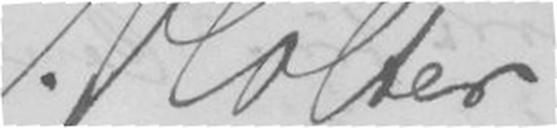Iver Holter
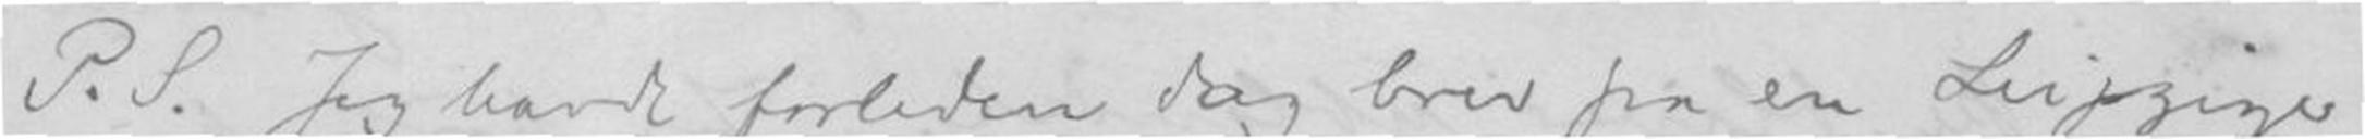P.S. jeg havde forleden dag brev fra en Leipziger
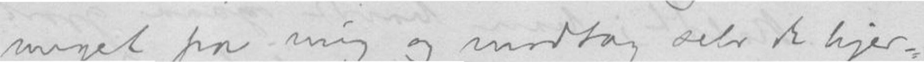meget fra mig og modtag selv de hjer-
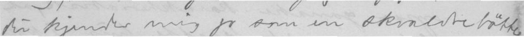du kjender mig jo som en skvaldrebøtte
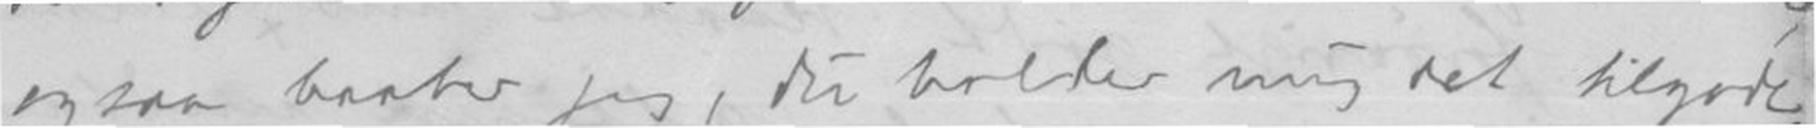og saa haaber jeg, du holder mig det tilgode
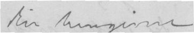Din hengivne
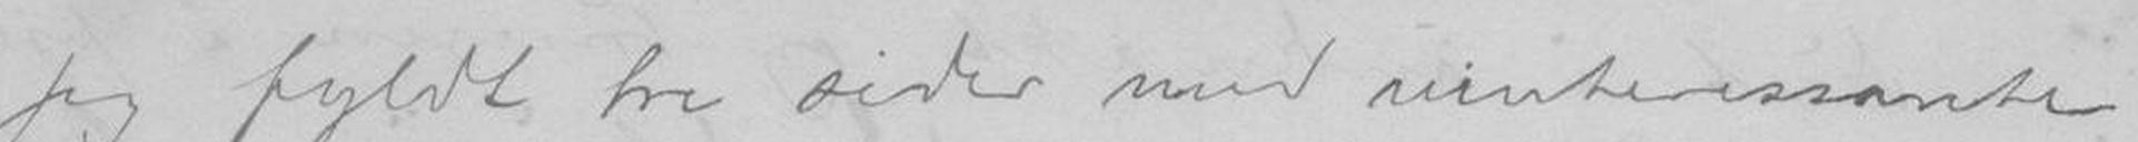jeg fyldt tre sider med uinteressante
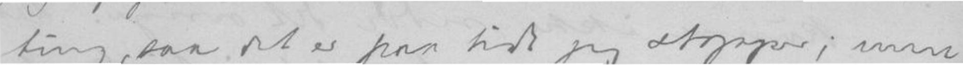ting, saa det er paa tide jeg stopper; men
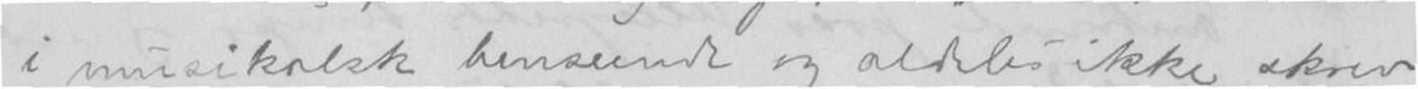i musikalsk henseende og aldeles ikke skrev
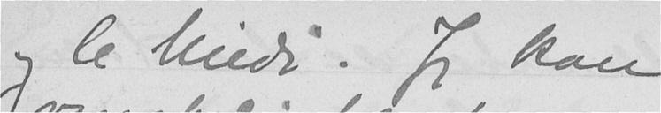og le Midi. Jeg kan
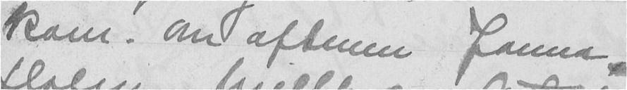kom. Om aftenen Janna,
